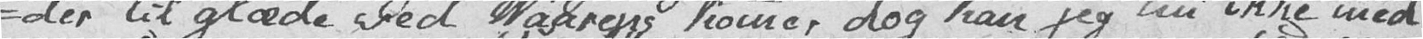-der til glæde ved Vaarens komme, dog kan jeg nu ikke med
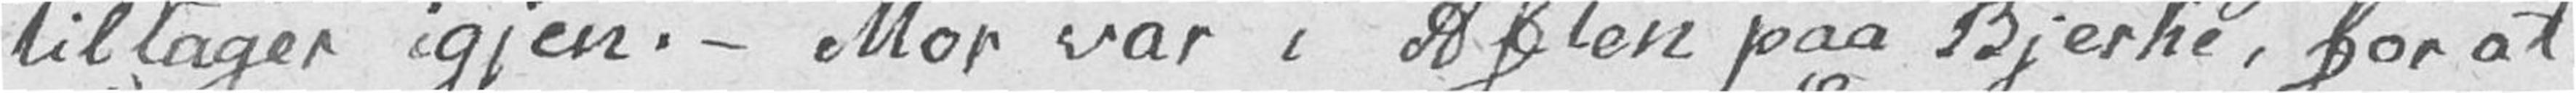tiltager igjen. – Mor var i Aften paa Bjerke, for at
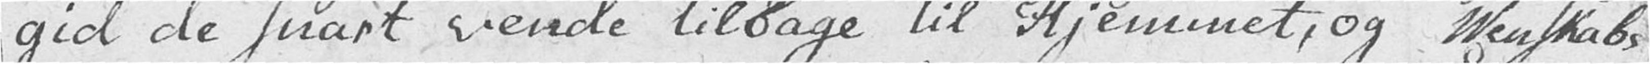gid de snart vende tilbage til Hjemmet, og Venskabs
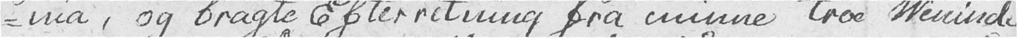-nia, og bragte Efterretning fra minne troe Veninder
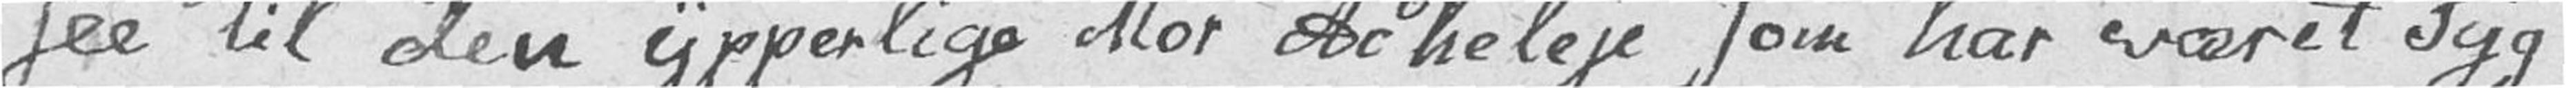see til den ypperlige Mor Acheleje som har været Syg
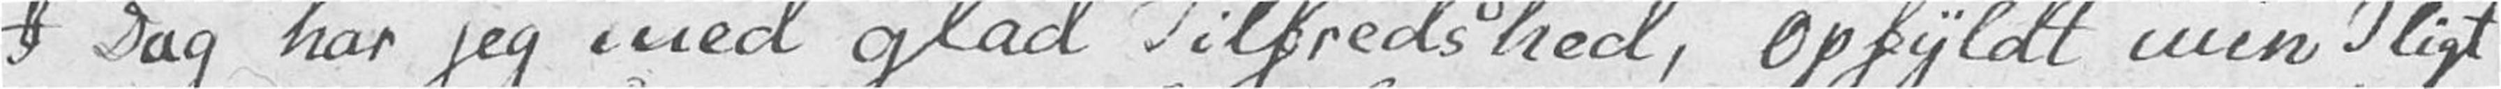I Dag har jeg med glad Tilfredshed, opfyldt min Pligt
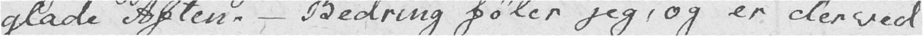glade Aften. – Bedring føler jeg, og er derved
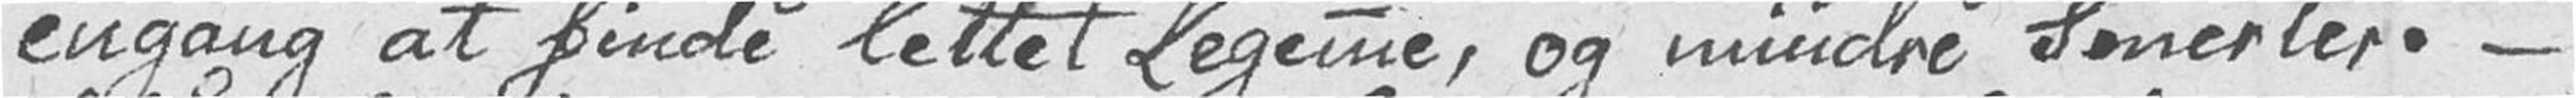engang at finde lettet Legeme, og mindre Smerter. –
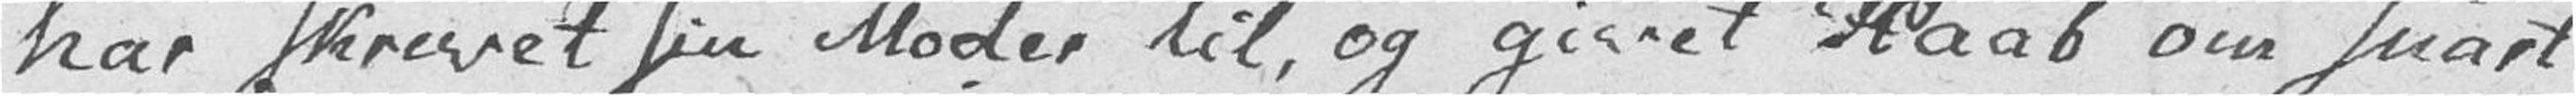har skrevet sin Moder til, og givet Haab om snart
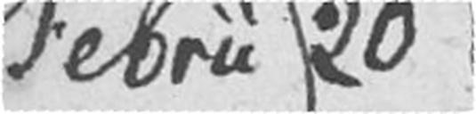Febru 20
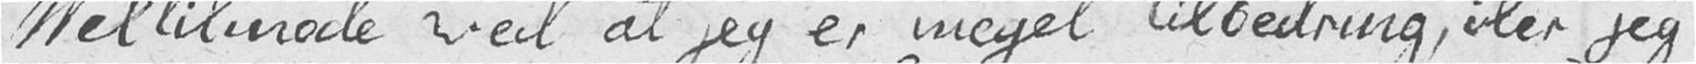Vel tilmode ved at jeg er meget til bedring, iler jeg
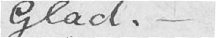Glad. –
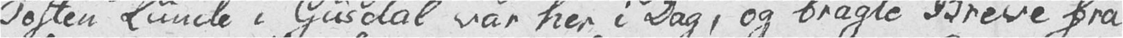Tosten Lunde i Gusdal var her i Dag, og bragte Breve fra
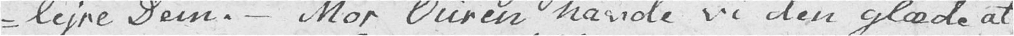-lejre Dem. – Mor Ouren havde vi den glæde at
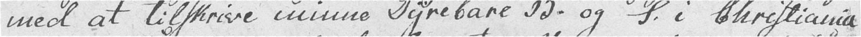med at tilskrive minne Dyrebare B. og S. i Christiania
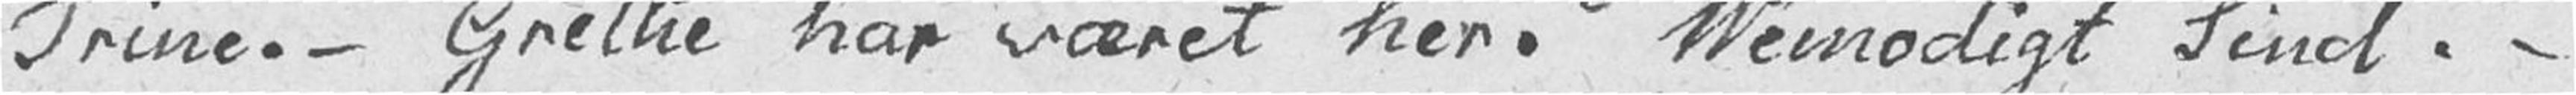Trine. – Grethe har været her. Vemodigt Sind. –
